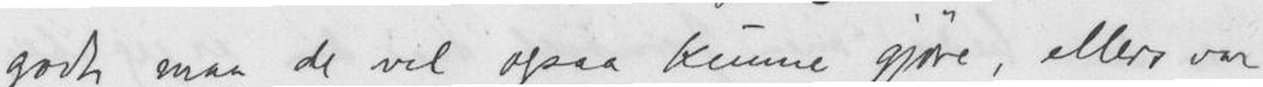godt maa de vil ogsaa kunne gjøre, eller var
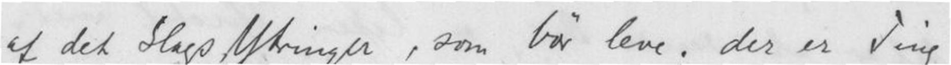af det Slags Ytringer, som bør leve; der er Ting
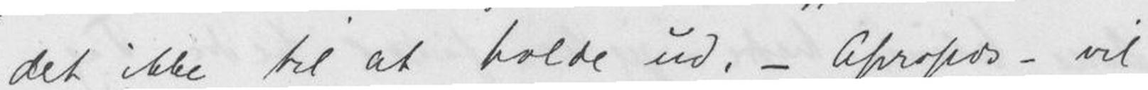det ikke til at holde ud,- Apropos - vil
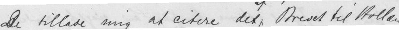De tillade mig at citere det, af Brevet til Hollæn-
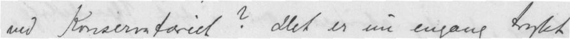ved Konservatoriet? Det er nu engang trykt
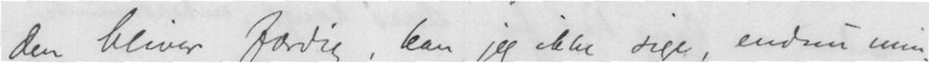den bliver Færdig, kan jeg ikke sige, endun min-
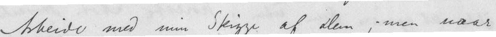Arbeide med min Skizze af Dem, men naar
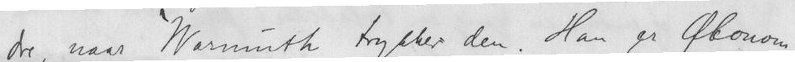dre, naar Warmuth trykker den. Han er Økonom
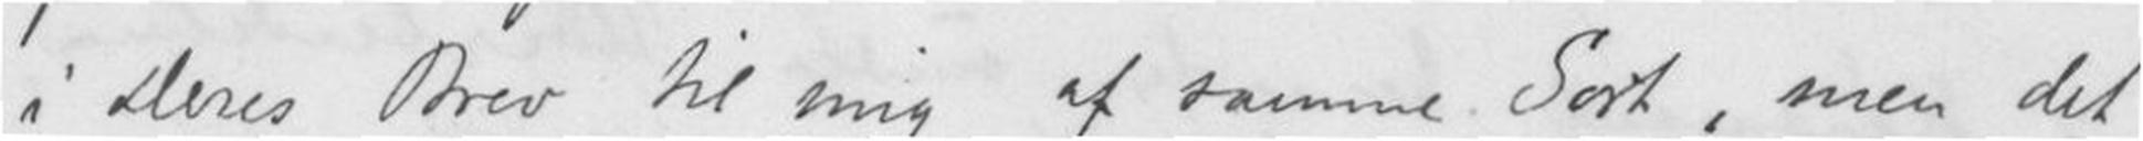i Deres Brev til mig af samme Sort, men det
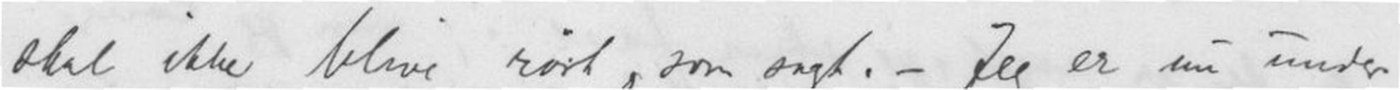skal ikke blive rært, som sagt.- Jeg er nu under
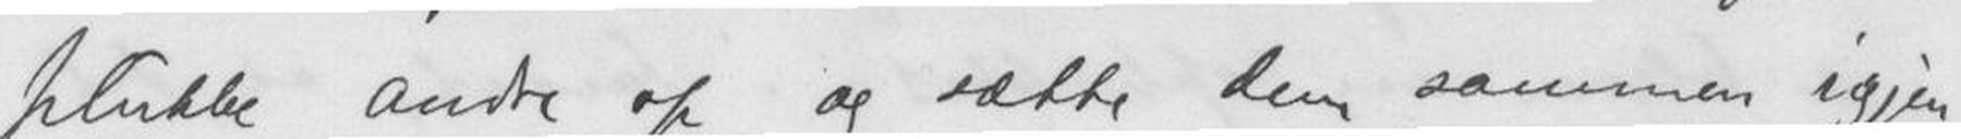plukke andre op og rætte dem sammen igjen,
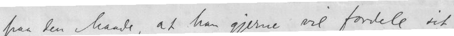paa den, maade, at han gjerne vil fordele sit
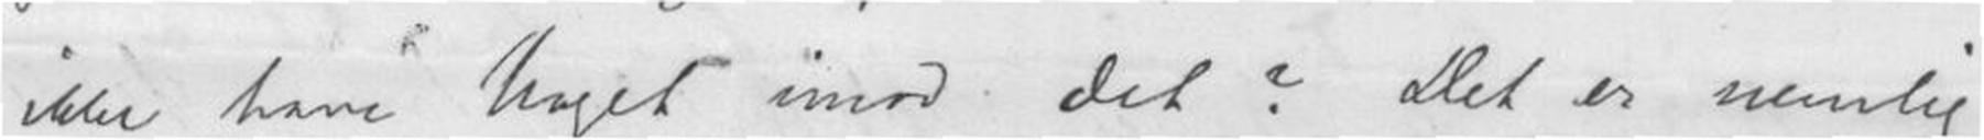ikke have byet imod det? Det er nemlig
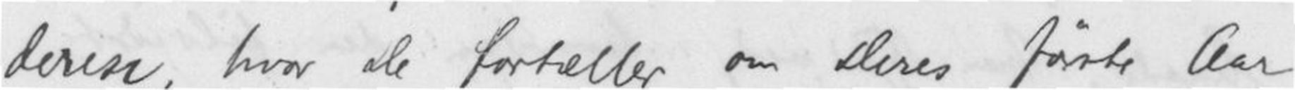deren, hvor de forteller om Deres første Aar
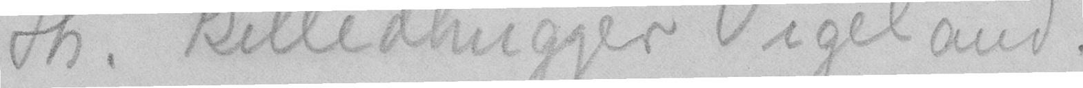Hr. Billedhugger Vigeland
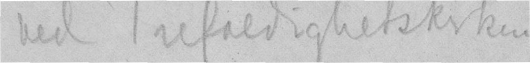ved Trefoldighetskirken
